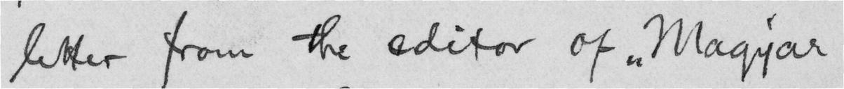letter from the editor of "Magyar
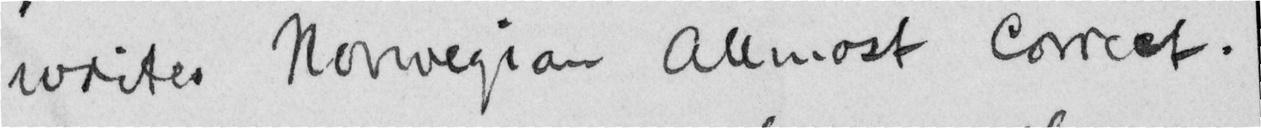writes Norwegian allmost correct.
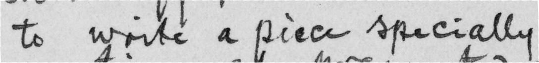to write a piece specially
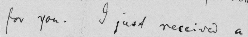for you. I just received a
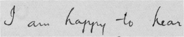I am happy to hear
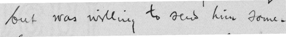but was willing to send him some-
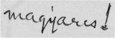magyares!
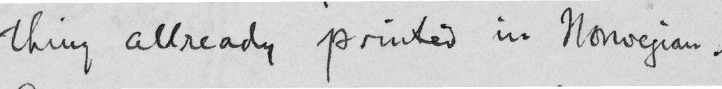thing allready printed in Norwegian.
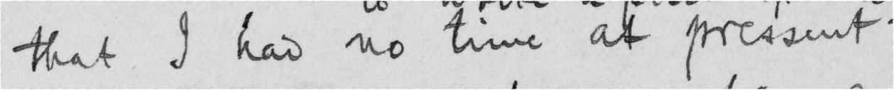that I had no time at pressent
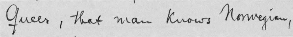Queer, that man knows Norwegian,
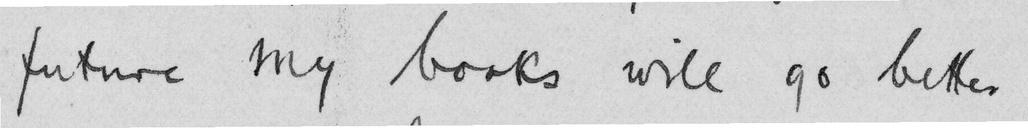future my books will go better
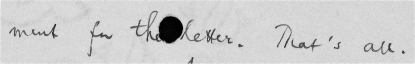ment for the letter. That's all.
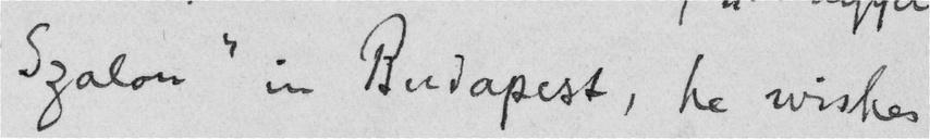Szalon" in Budapest, he wishes
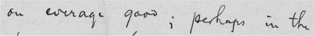on everage good; perhaps in the
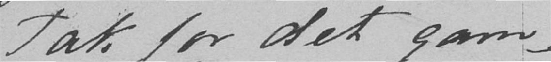Tak for det gam-
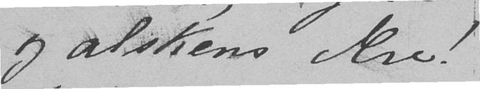og alskens Ære!
